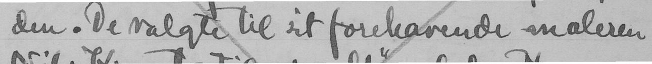den. De valgte til sit forehavende maleren
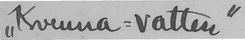"Kvenna-vatten"
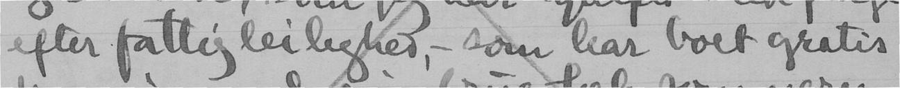efter fattig leilighed, - som har boet gratis
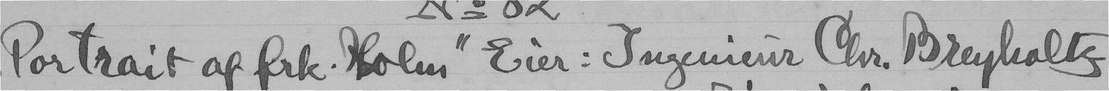"Portrait af frk Holm" Eier: Ingenieur Chr. Breyholtz
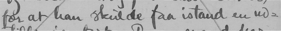for at han skulde faa istand en ud-
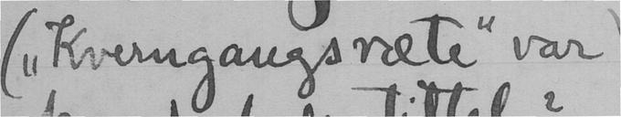("Kverngangsvæte" var
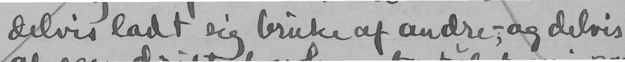delves ladt sig bruke af andre, - og delvis
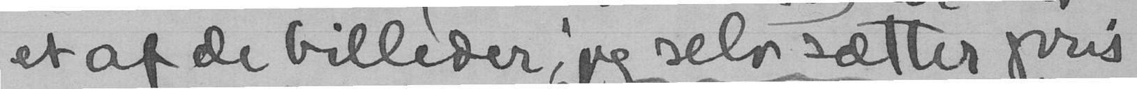et af de billeder, jeg selv sætter pris
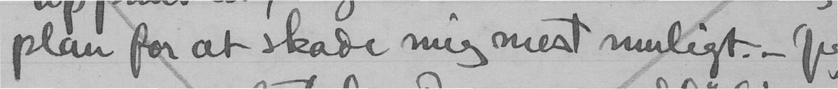plan for at skade mig mest muligt. - Jeg
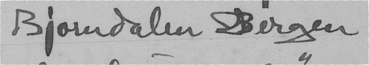Bjørndalen Bergen
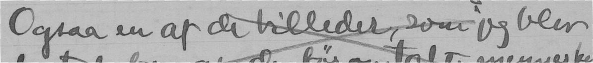Ogsaa en af de billeder, som jeg blev
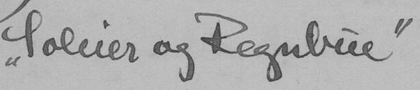"Soleier og Regnbue"
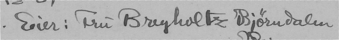Eier: Fru Breyholtz Bjørndalen
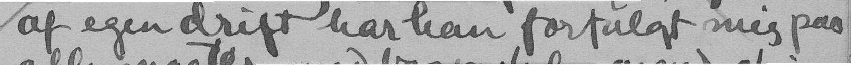af egen drift har han forfulgt mig paa
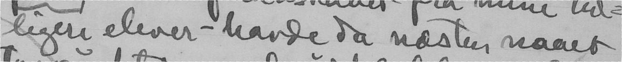ligere elever - havde da næsten naaet
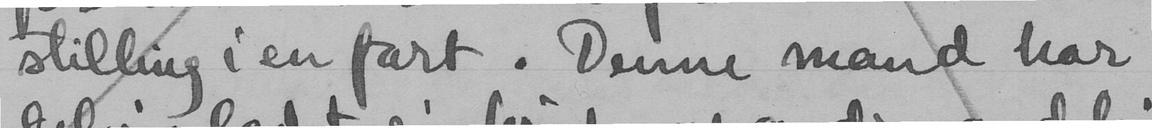stilling i en fart. Denne mand har
